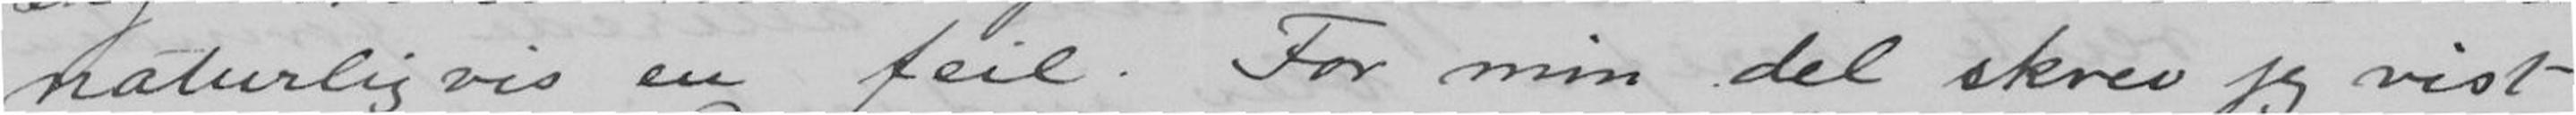naturligvis en feil. For min del skrev jeg vist
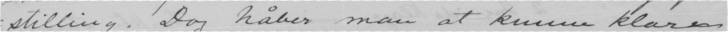stilling. Dog håber man at kunne klare
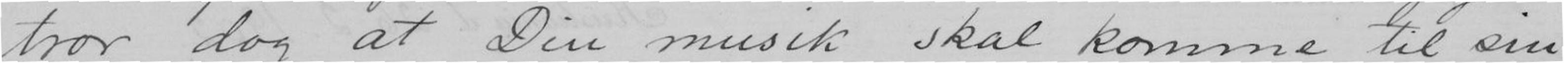tror dog at Din musik skal komme til sin
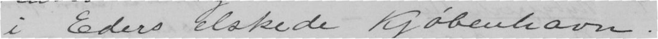i Eders elskede Kjøbenhavn.
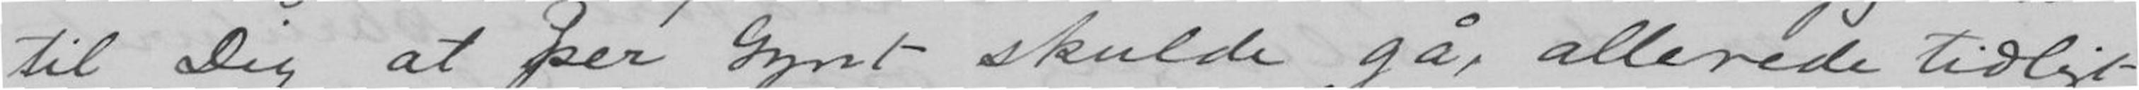til Dig at Per Gynt skulde gå, allerede tidligt
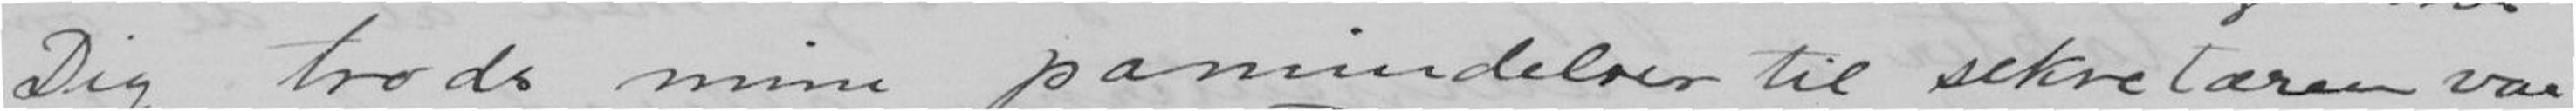Dig trods mine påmindelser til sekretæren var
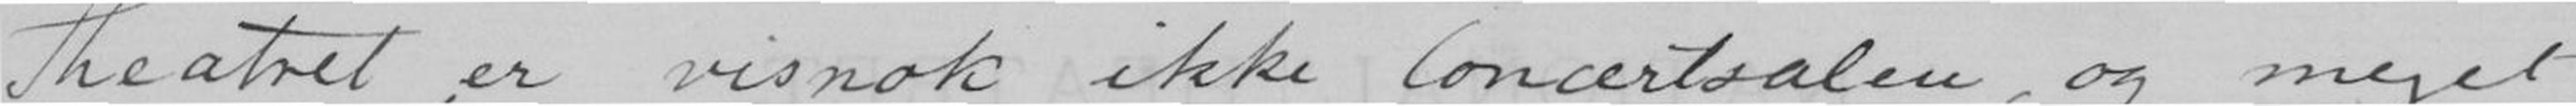Theatret er vistnok ikke Concertsalen, og meget
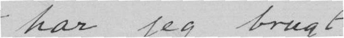har jeg brugt
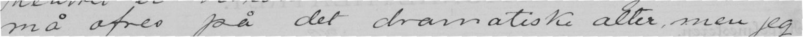må ofres på det dramatiske alter, men jeg
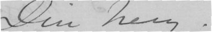Din heng.
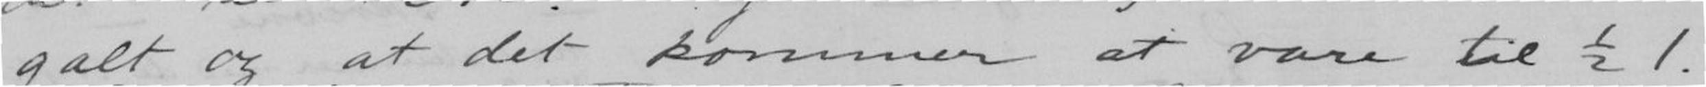galt og at det kommer at vare til ½1.
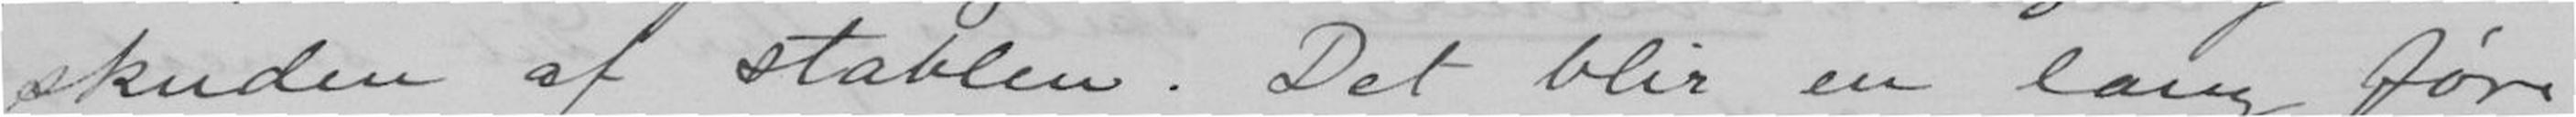skuden af stablen. Det blir en lang føre-
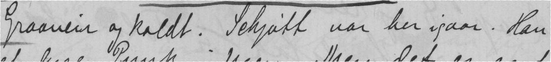Graaveir og kaldt. Schjøtt var her igaar. Han
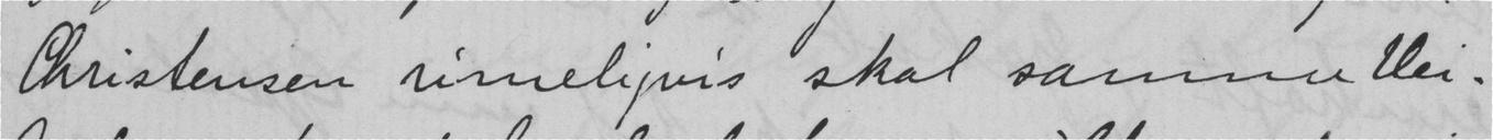Christensen rimeligvis skal samme vei.
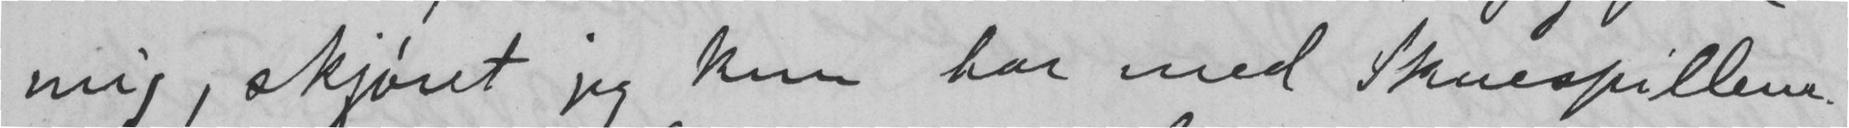mig, skjønt jeg kun har med Skuespillene.
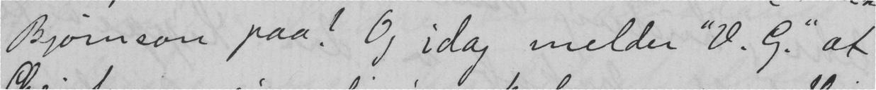Bjørnson paa! Og idag melder "V. G." at
